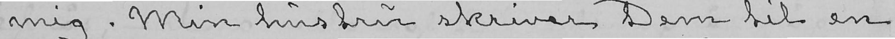mig. Min hustru skriver Dem til en
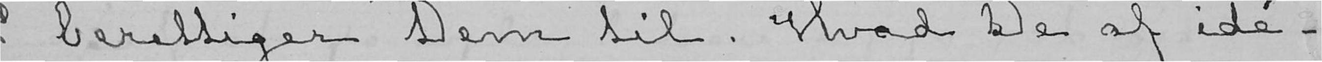berettiger Dem til. Hvad De af idé-
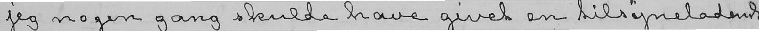jeg nogen gang skulde have givet en tilsyneladende
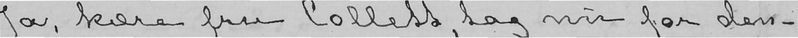Ja, kære fru Collett, tag nu for den-
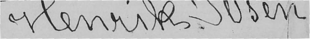Henrik Ibsen.
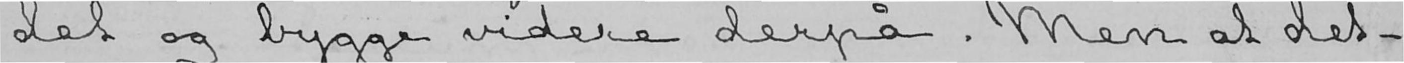det og bygge videre derpå. Men at det-
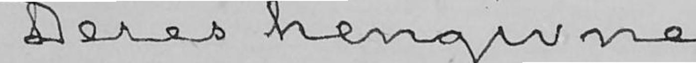Deres hengivne
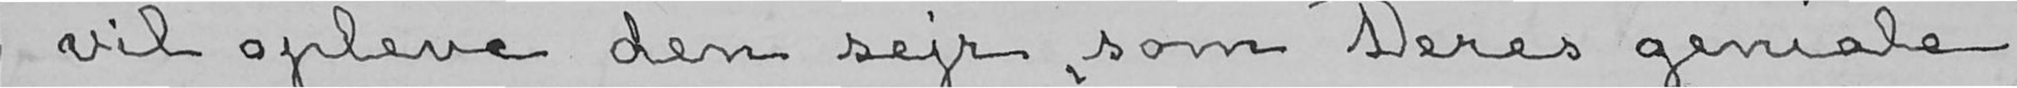vil opleve den sejr, som Deres geniale
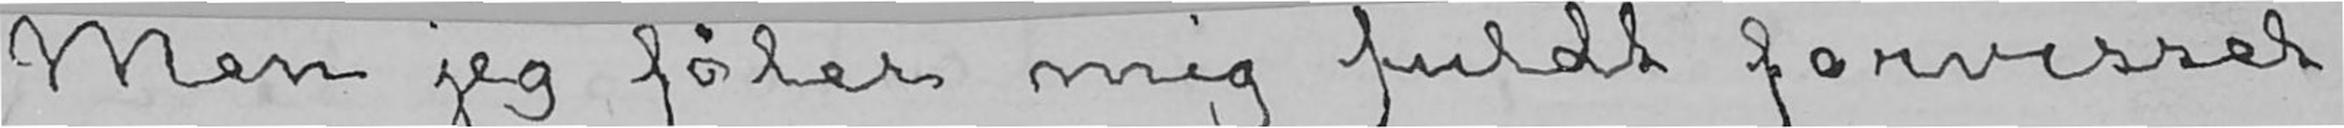Men jeg føler mig fuldt forvisset
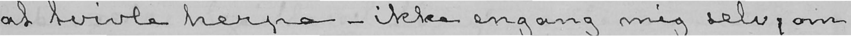at tvivle herpå- ikke engang mig selv, om
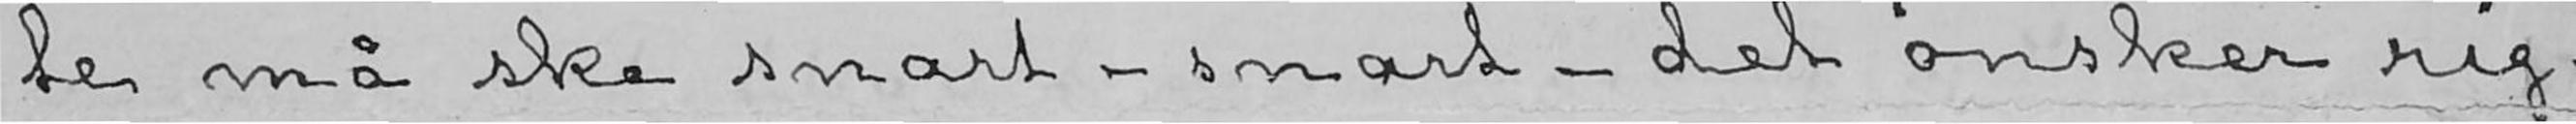te må ske snart- snart- det ønsker rig-
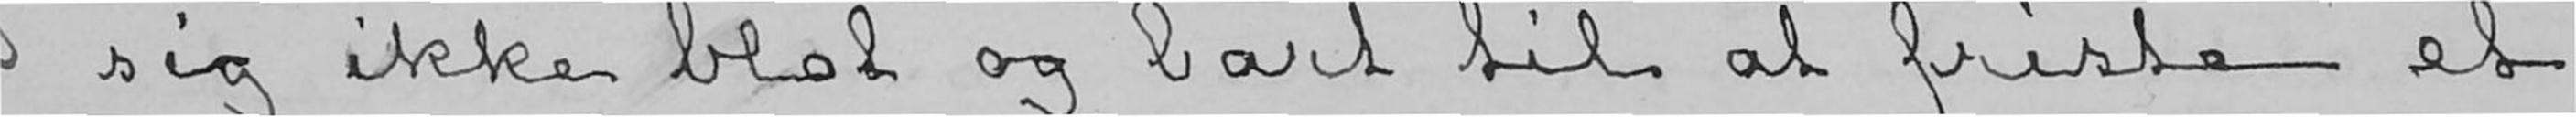sig ikke blot og bart til at friste et
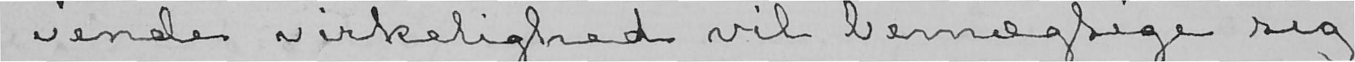vende virkelighed vil bemægtige sig
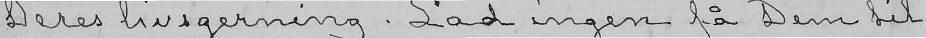Deres livsgerning. Lad ingen få Dem til
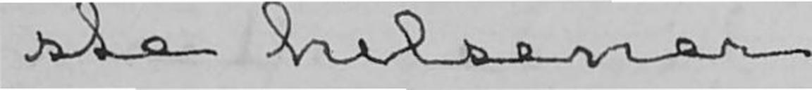ste hilsener
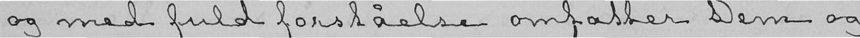og med fuld forståelse omfatter Dem og
